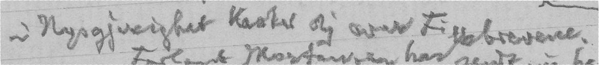i Nysgjerrighet kaster dig over Fillebrevene.
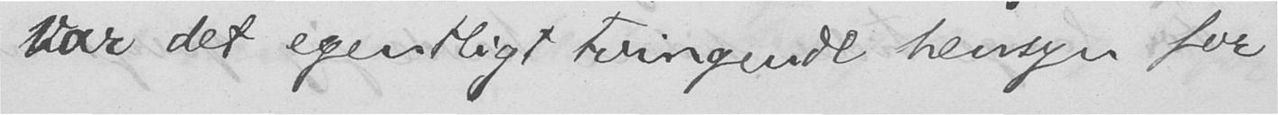var det egentligt tvingende hensyn for
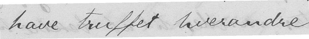have truffet hverandre.
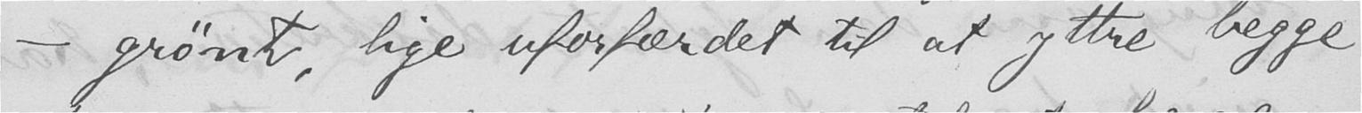– grønt, lige uforfærdet til at yttre begge
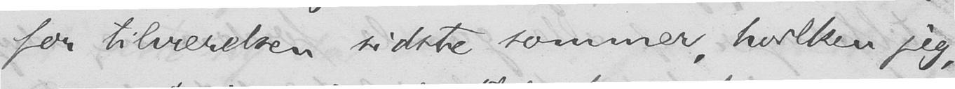for tilværelsen sidste sommer, hvilken jeg,
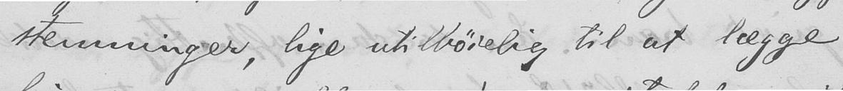stemninger, lige utilbøielig til at lægge
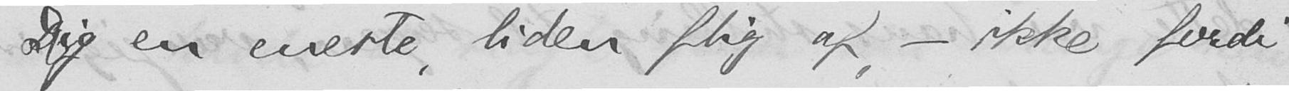Dig en eneste, liden flig af, - ikke fordi
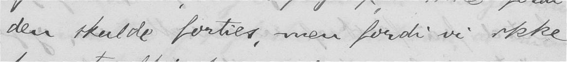den skulde forties, men fordi vi ikke
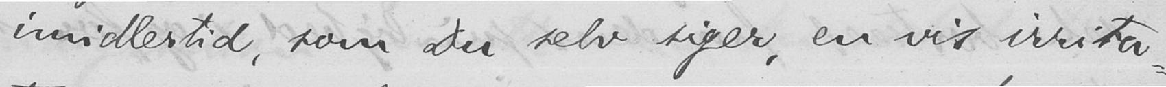imidlertid, som Du selv siger, en vis irrita-
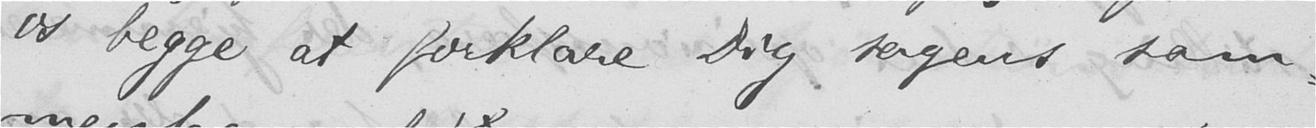os begge at forklare Dig sagens sam-
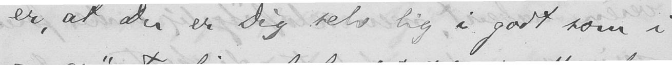er, at Du er Dig selv lig i godt som i
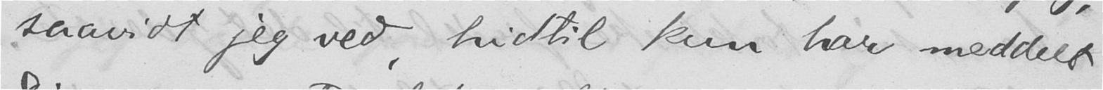saavidt jeg ved, hidtil kun har meddelt
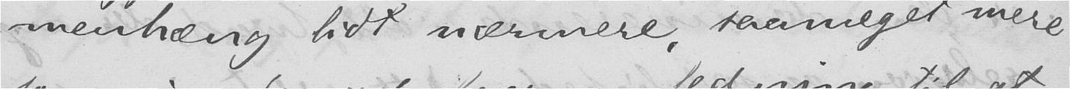menhæng lidt nærmere, saameget mere
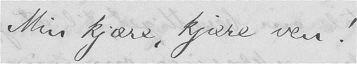Min kjære, kjære ven!
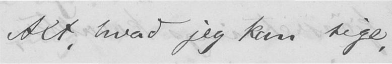Alt, hvad jeg kan sige,
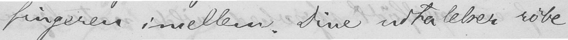fingeren imellem. Dine udtalelser røbe
